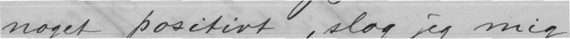noget positivt, slog jeg mig
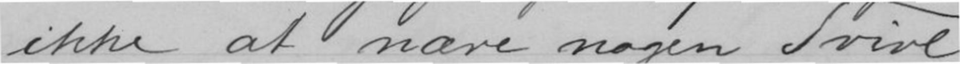tikke at nære nogen Tvivl
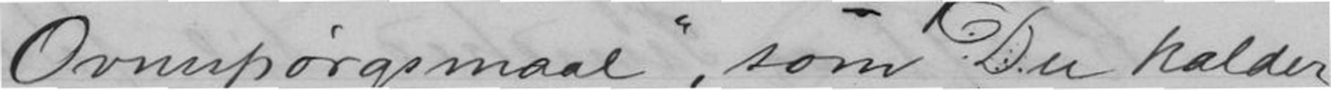Ovnespørgsmaal, som Du kalder
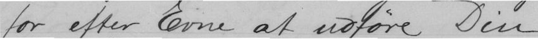for efter Evne at udføre Din
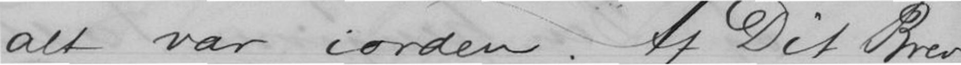alt var iorden. At Dit Brev
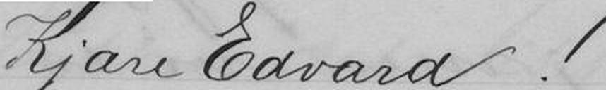Kjære Edvard!
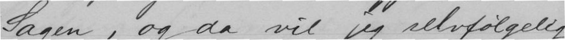Sagen, og da vil jeg selvfølgelig
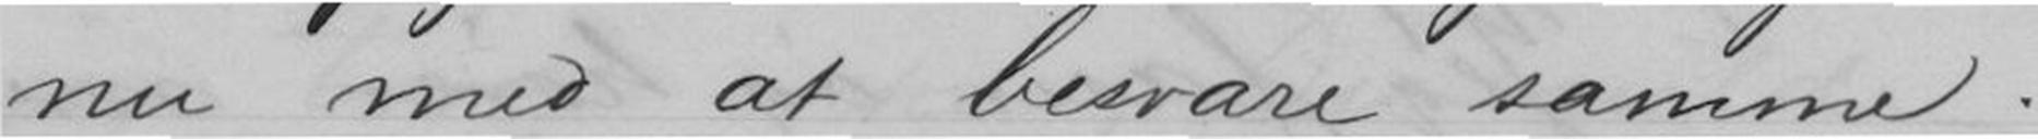nu med at besvare samme.
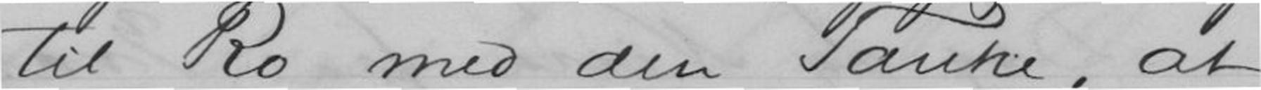til Ro med den Tanke, at
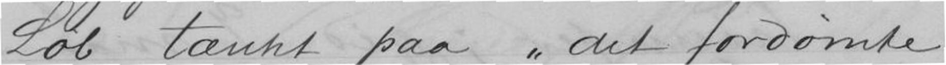Sol tænkt paa det fordømte
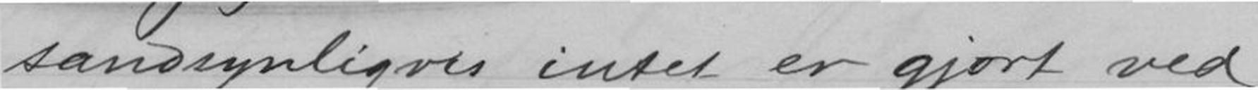sandsynligvis intet er gjort ved
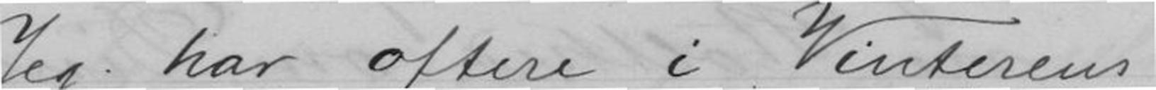Jeg har oftere i Vinterens
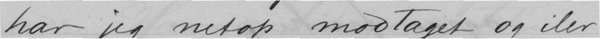har jeg netop modtaget og iler
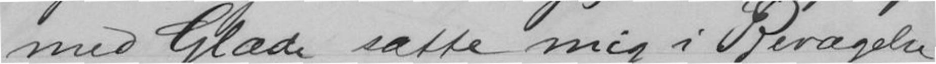med Glæde sætte mig i Bevægelse
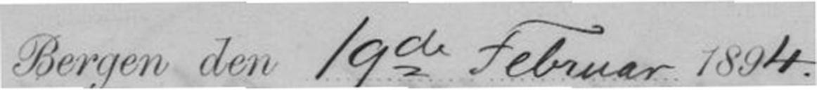Bergen den 19de Februar 1894
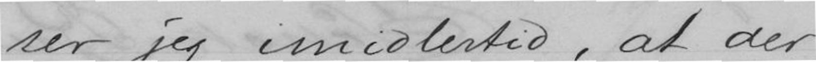ser jeg imidlertid, at der
